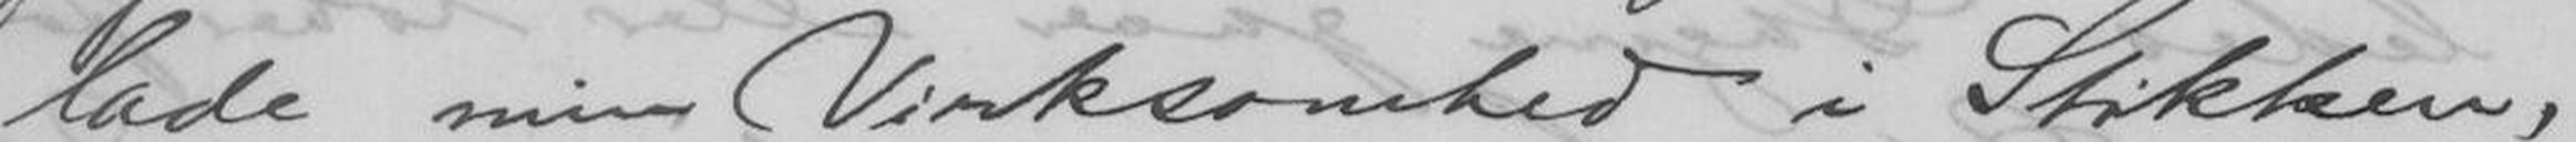lade min Virksomhed i Stikken,
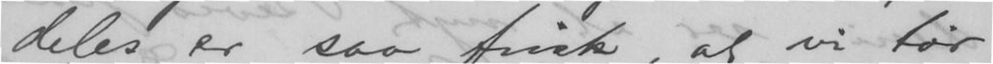deles er saa frisk, at vi tør
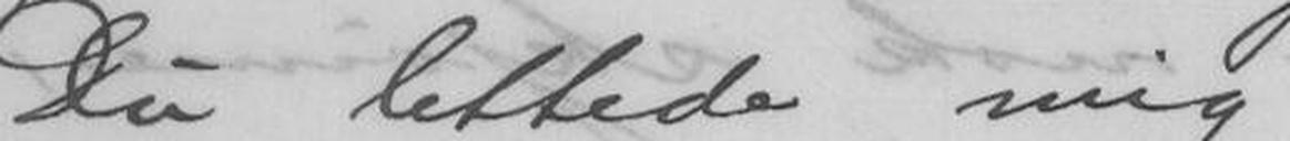Du lettede mig. -
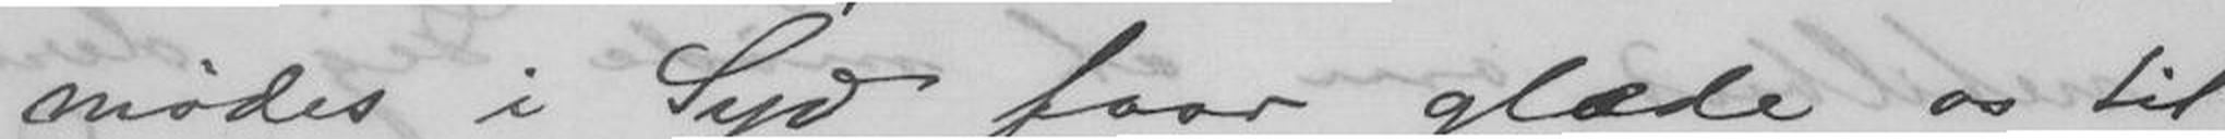mødes i Syd faar glæde os til
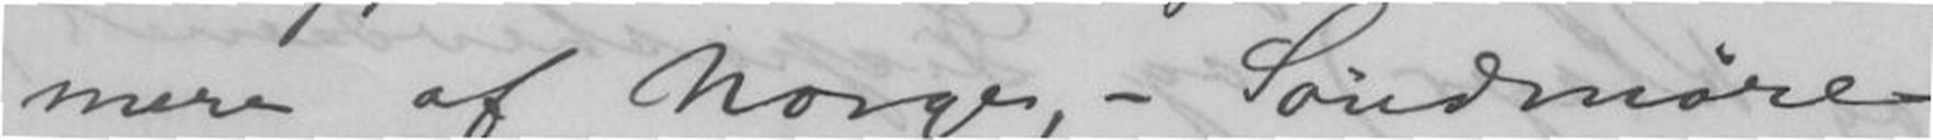mere af Norge, - Søndmøre
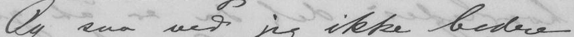Og saa ved jeg ikke bedre
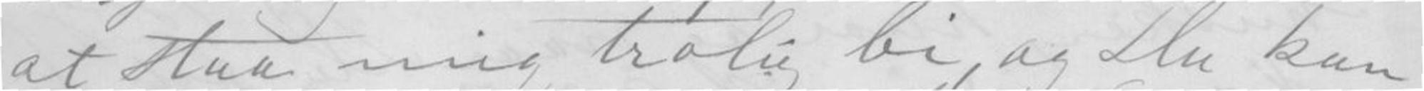at staa mig trolig bi, og Du kan
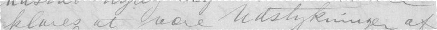klares at være Udstykninger af
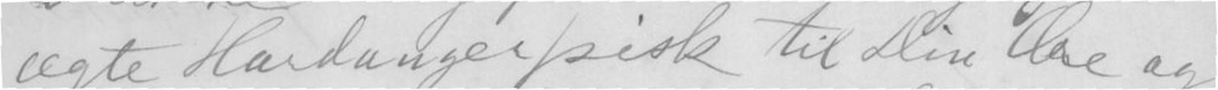ægte Hardangerpisk til Din Ære og
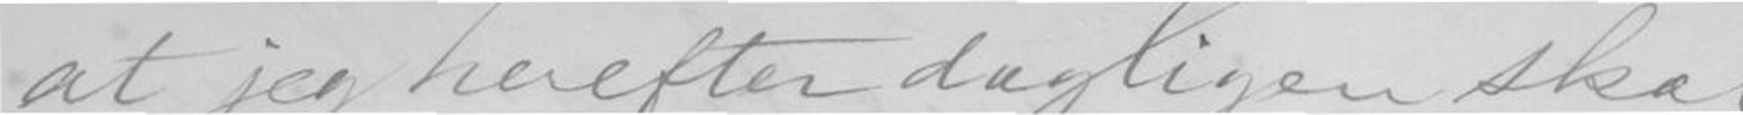at jeg herefter dagligen skal
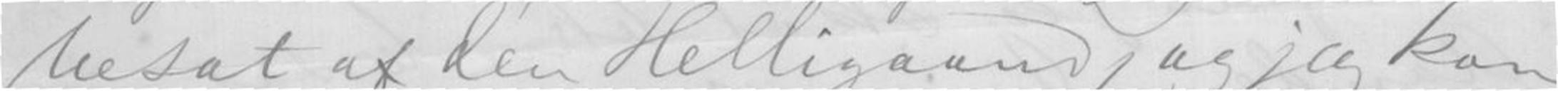besat af den Helligaand, og jeg kan
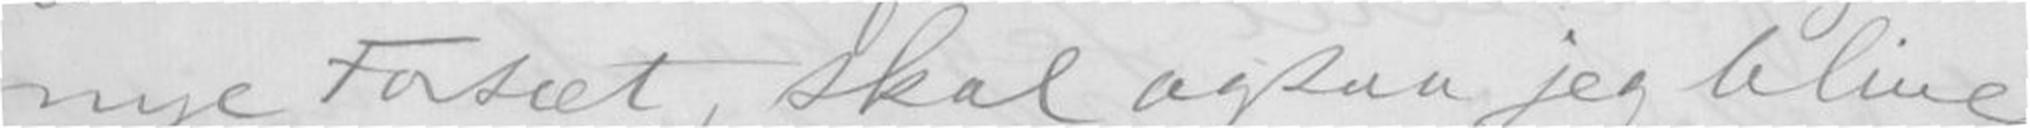nye Forsæt, skal ogsaa jeg blive
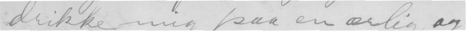drikke mig paa en ærlig og
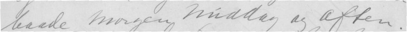baade Morgen Middag og Aften.
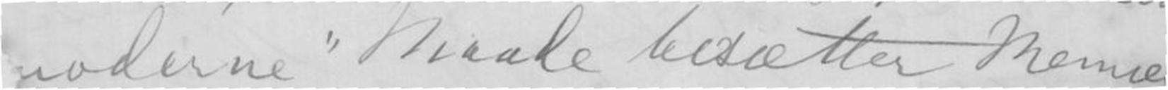moderne "Maade besætter Menne
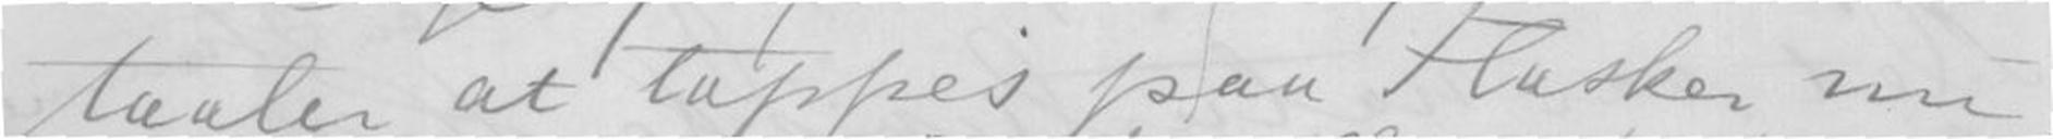taaler at tappes paa Flasken nu
